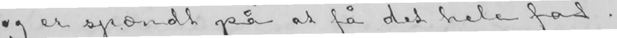og er spændt på at få det hele fat.
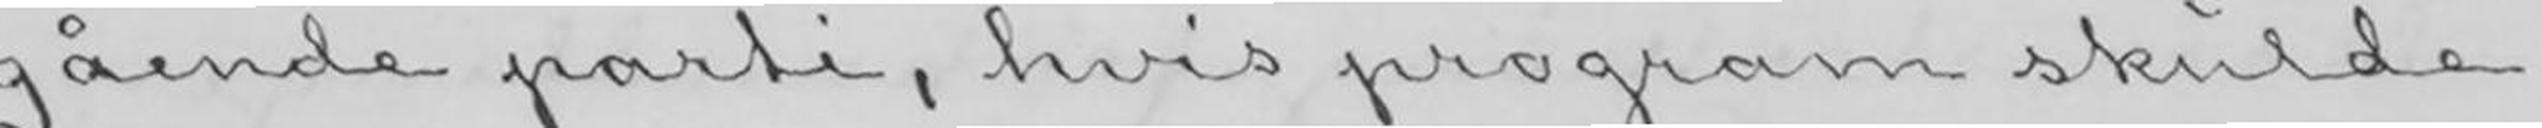gående parti, hvis program skulde
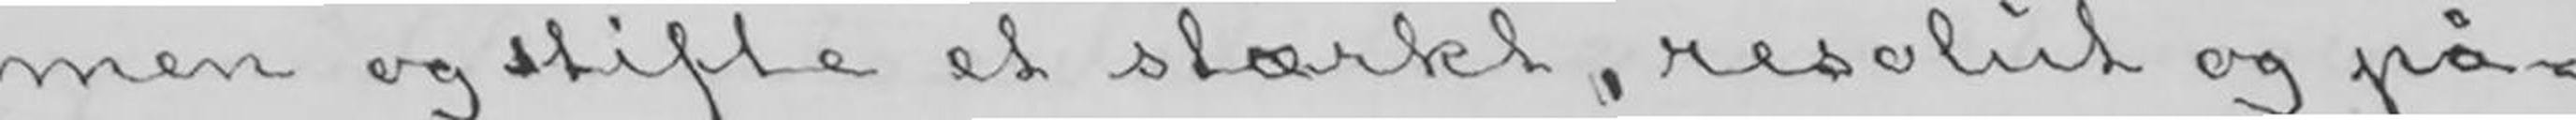men og stifte et stærkt, resolut og på-
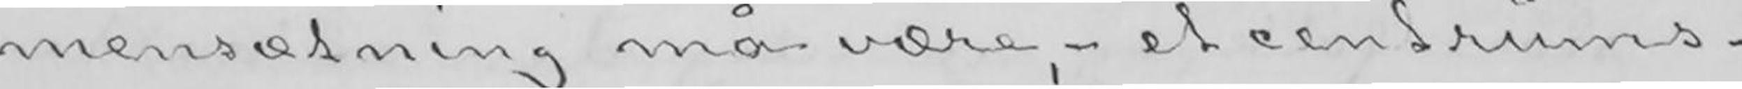mensætning må være,- et centrums-
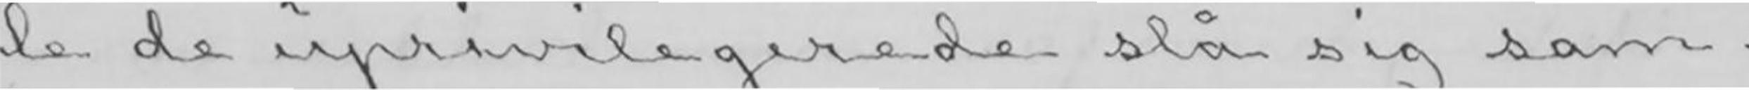le de uprivilegerede slå sig sam-
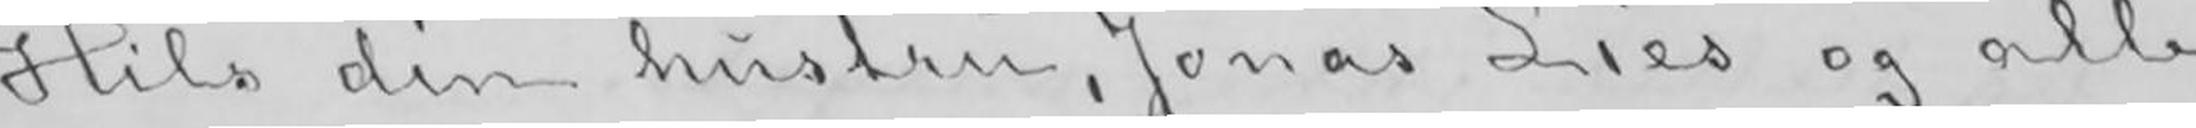Hils din hustru, Jonas Lies og alle
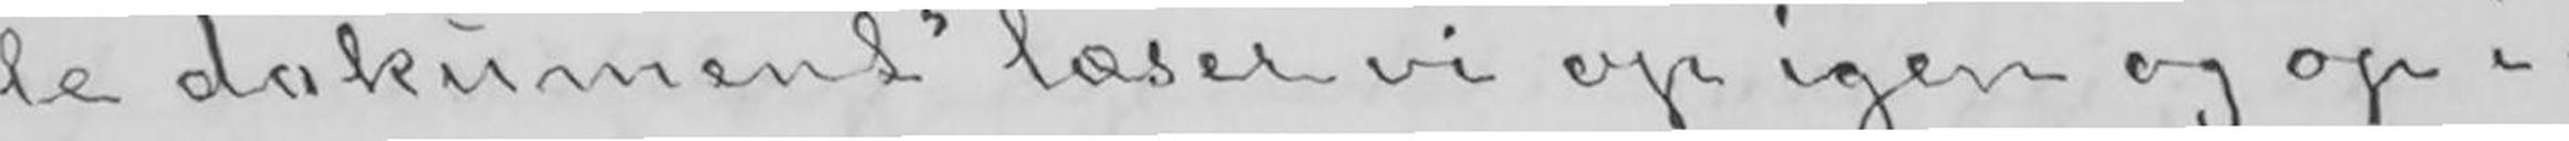le dokument» læser vi op igen og op i-
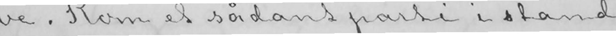ve. Kom et sådant parti i stand,
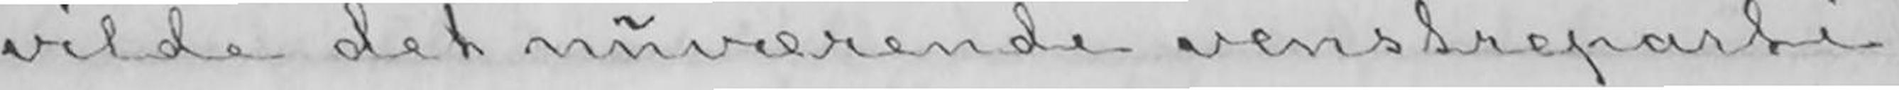vilde det nuværende venstreparti
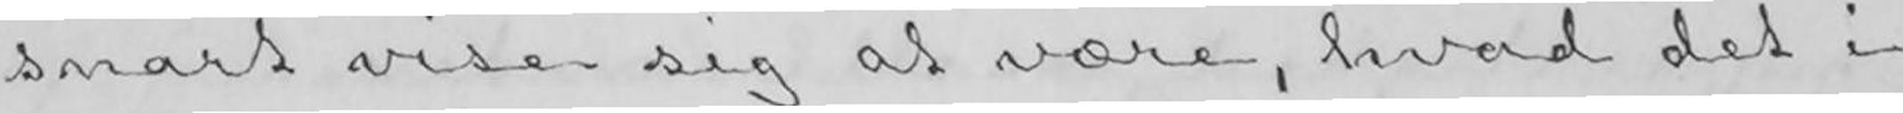snart vise sig at være, hvad det i
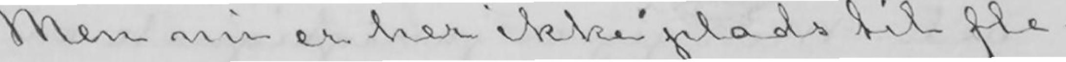Men nu er her ikke plads til fle-
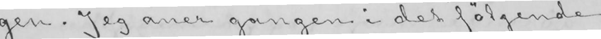gen. Jeg aner gangen i det følgende
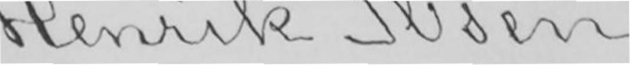Henrik Ibsen.
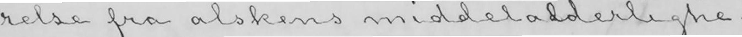relse fra alskens middelalderlighe-
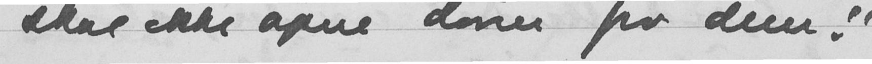skal ikke åpne dører for dem."
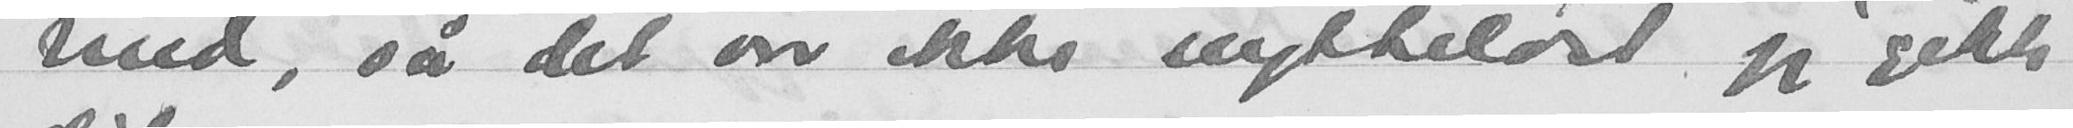med, så det var ikke nytteløst, jeg gikk
